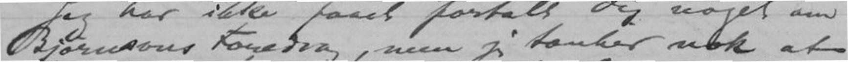Bjørnsons Foredrag, men jeg tænker nok at
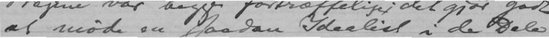at møde en saadan Idealist i de Dele
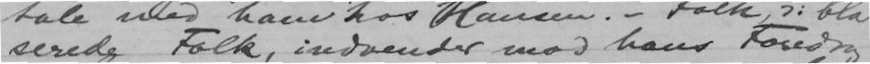serede Folk, indvender mod hans Foredrag
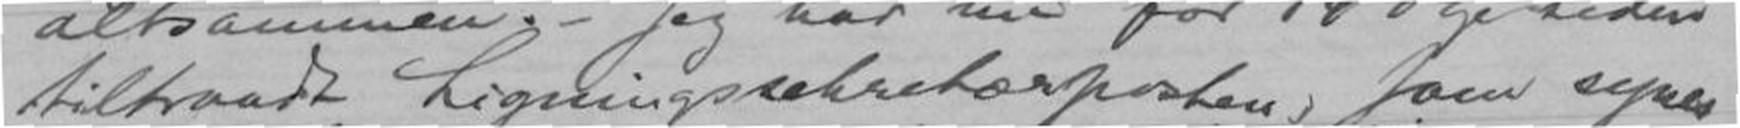tiltraadt Ligningssekretærposten, som synes
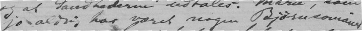jo aldrig har været nogen Bjørnsonianer,
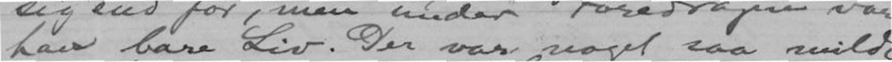han bare Liv. Der var noget saa mildt
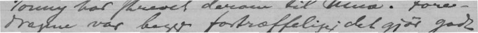dragene var begge fortræffelige; det gjør godt
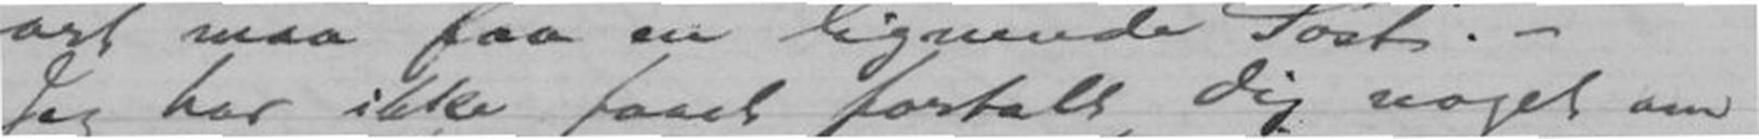Jeg har ikke faaet fortalt dig noget om
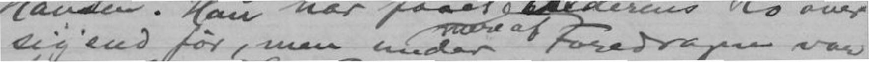sig end før, men under Foredragene var
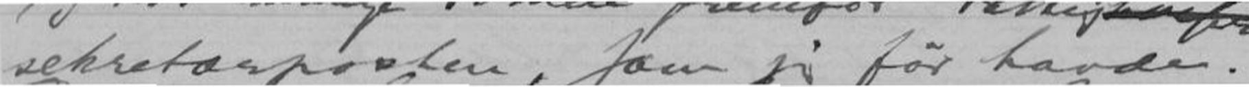sekretærposten, som jeg før havde.
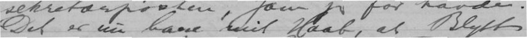Det er nu bare mit Haab, at Blytt
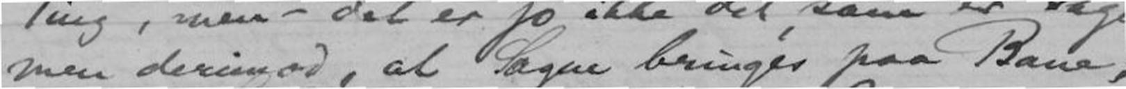men derimod, at Sagen bringes paa Bane,
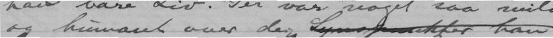og humant over den Synspunkter han
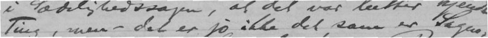Ting, men - det er jo ikke det, som er Sagen,
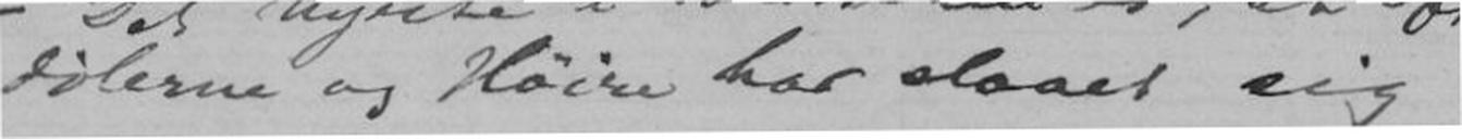dølerne og Høire har slaaet sig
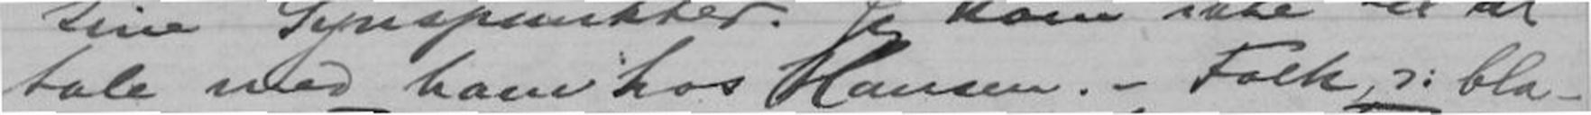tale med ham hos Hansen. - Folk, ): bla-
